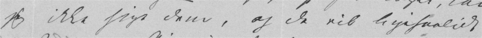jeg ikke sige Dem, og De vil ligesaalidt
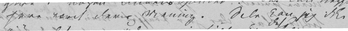have været Deres Mening. Selv kan jeg ikke
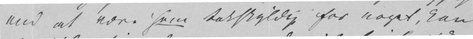end at være ham takskyldig for noget, kan
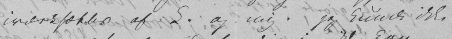iværksættes af C. og mig. jeg kunde ikke
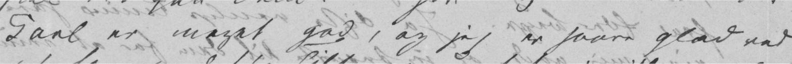Carl er meget god, og jeg er saare glad ved
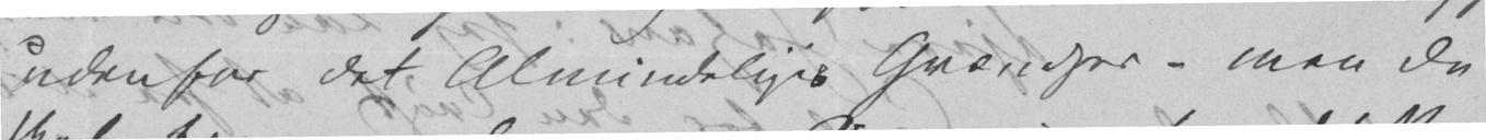udenfor det Almindeliges Grændser – men De
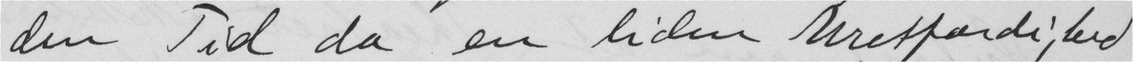den Tid da en liden Uretfærdighed
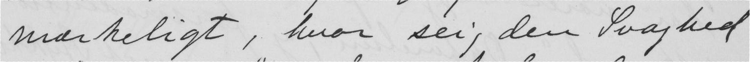mærkeligt, hvor seig den Svaghed
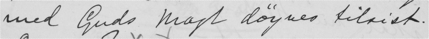med Guds Magt døyves tilsist.
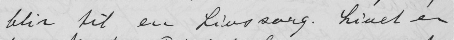blir til en Livssorg. Livet er
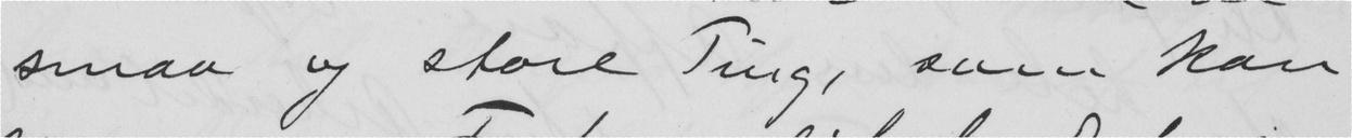smaa og store Ting, som kan
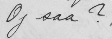Og saa?
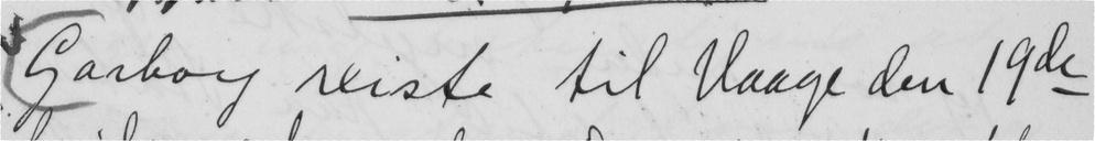Garborg reiste til Vaage den 19de
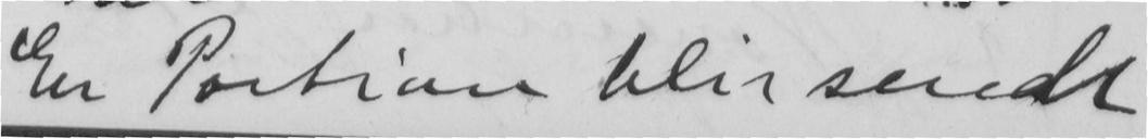En Portion blir sendt
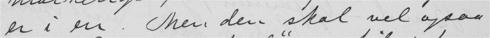er i en. Men den skal vel ogsaa
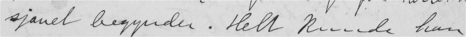sjauet begynder. Helt kunde han
